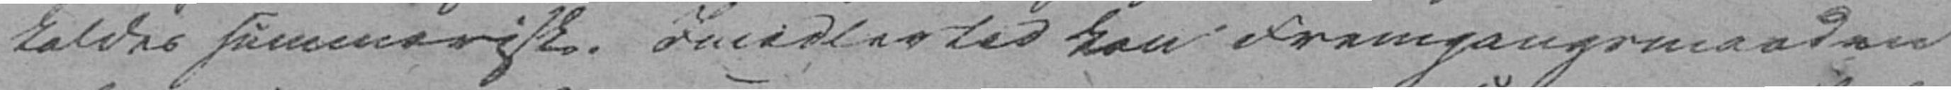kaldes summarisk. Imidlertid kan Fremgangsmaaden
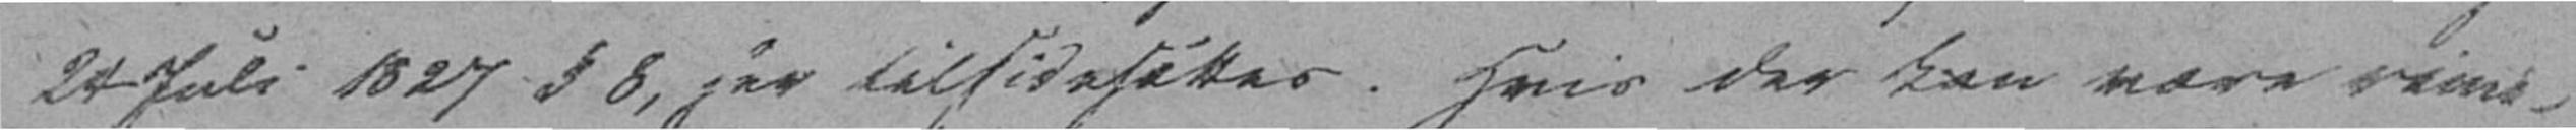24 Juli 1827 § 8, her tilsidesættes. Hvis der kan være rime-
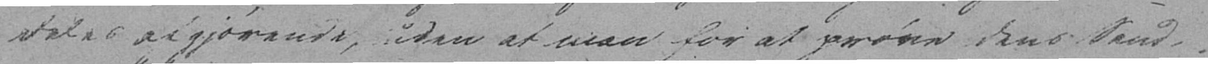deles afgjørende, uden at man for at prøve dens Sand-
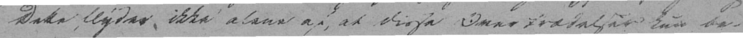Dette flyder ikke alene af, at disse Overtrædelser kun be-
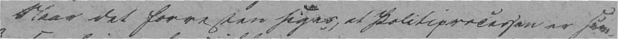Naar det forresten siges, at Politiprocessen er sum-
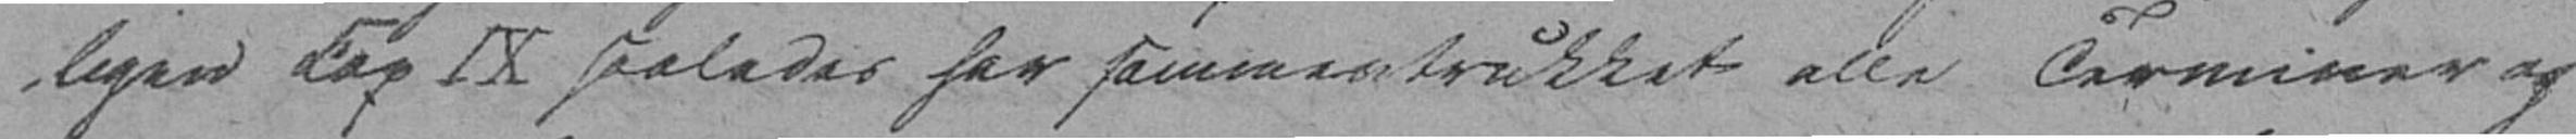ligen Cap IX saaledes har sammentrukket alle Terminer og
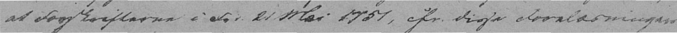at Forskrifterne i Frd. 21 Mai 1757, cfr. disse Forelæsningers
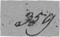359.
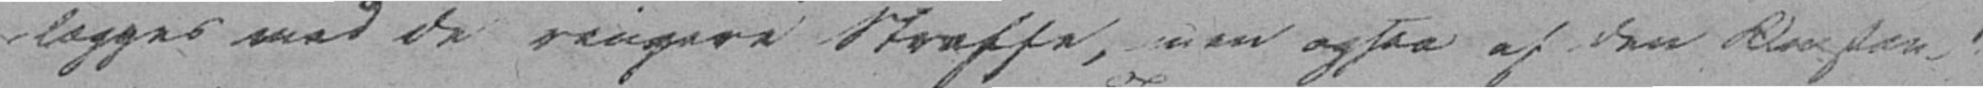lægges med de ringere Straffe, men ogsaa af den Omstæn-
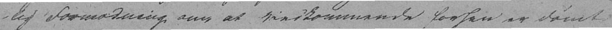lig Formodning om, at vedkommende forhen er dømt
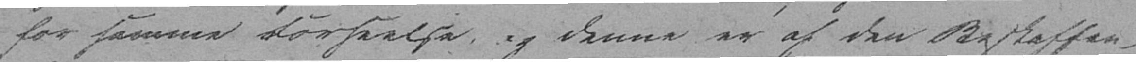for samme Forseelse, og denne er af den Beskaffen-
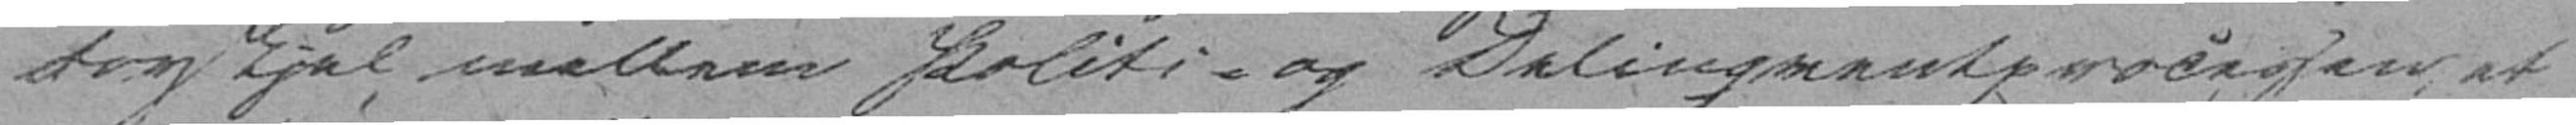Forskjel mellem Politi- og Delinqventprocessen, at
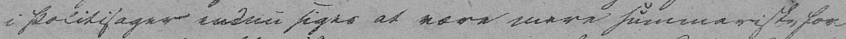i Politisager endnu siges at være mere summarisk, for-
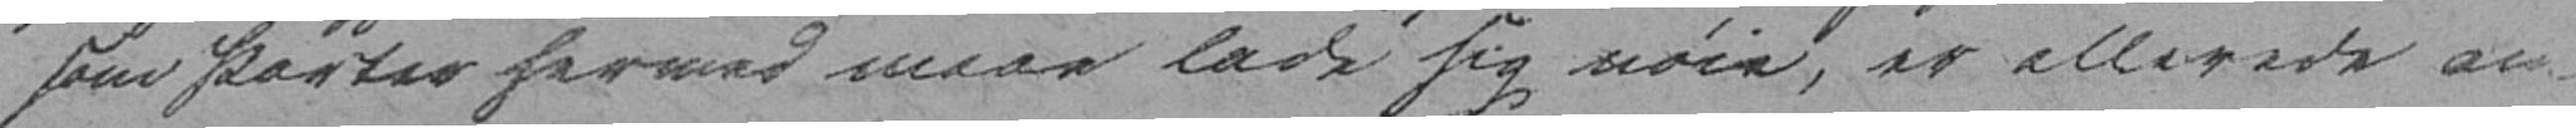som Parter hermed maae lade sig nøie, er allerede an-
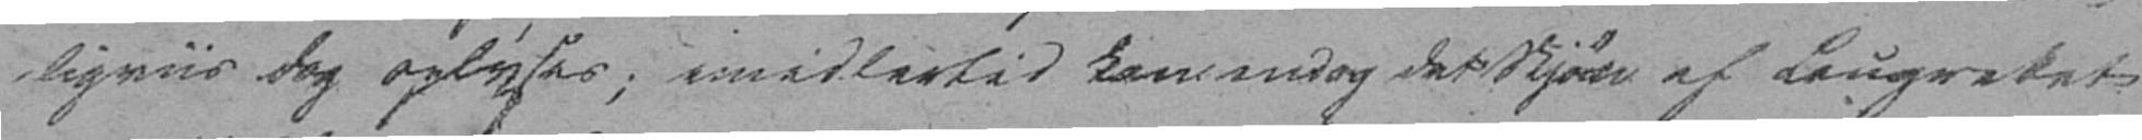ligviis dog oplyses; imidlertid kan endog det Skjøn af Laugrettet
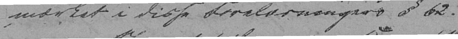mærket i disse Forelæsningers § 62.
